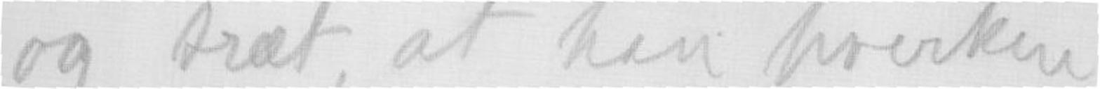og træt, at han hverken
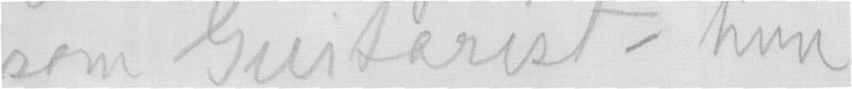som Guitarist hun
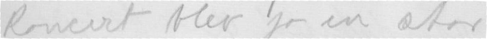Koncert blev jo en stor
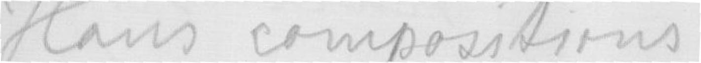Hans Compositions-
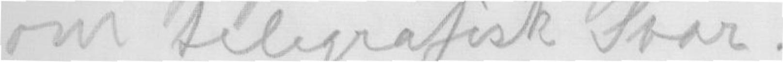om telegrafisk Svar.
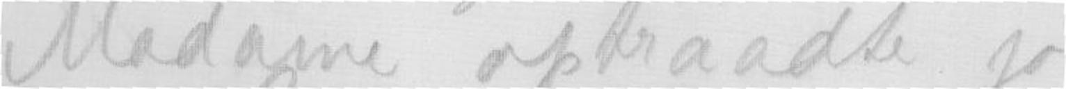Madame optraadte jo
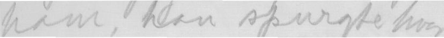ham, han spurgte hvor-
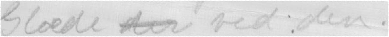Glæde der ved den.
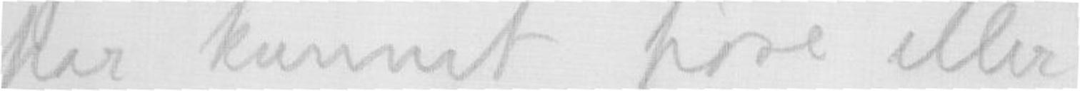har kunnet høre eller
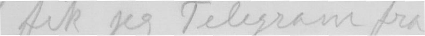fik jeg Telegram fra
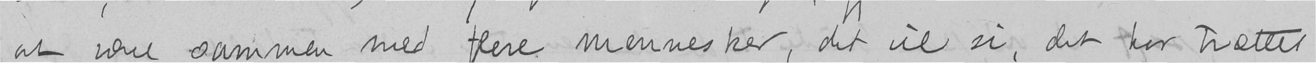at være sammen med flere mennesker, det vil si, det har trættet
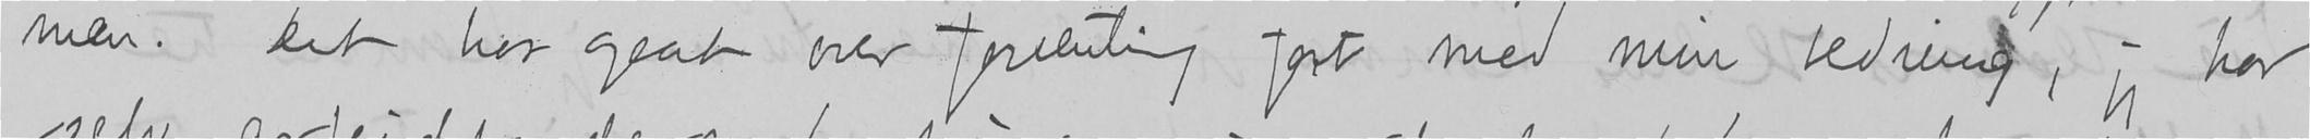men. Det har gaat over forventning fort med min bedring, jeg har
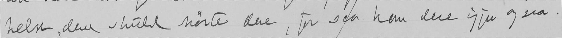helst, dere skulde hørte dere, for saa kom dere igjen ogsaa.
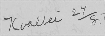Kvalbei 27/8.
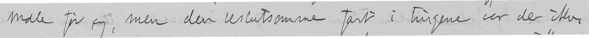male før og, men den beslutsomme fart i tingene var der ikke,
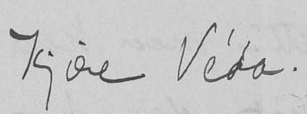Kjære Véda.
